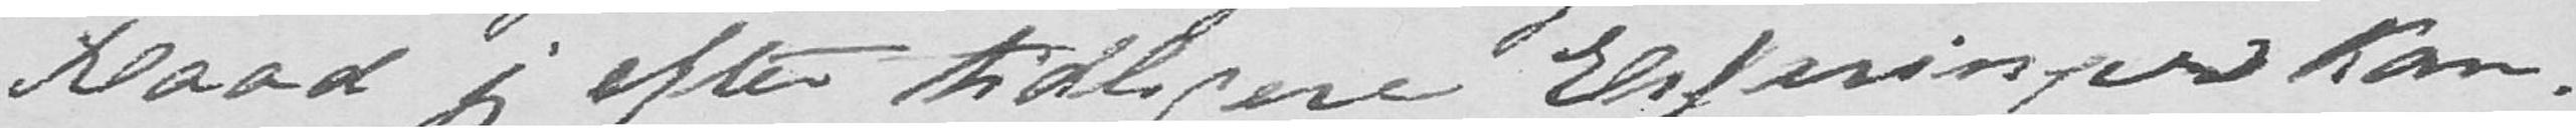Raad jeg efter tidligere Erferingen kan.
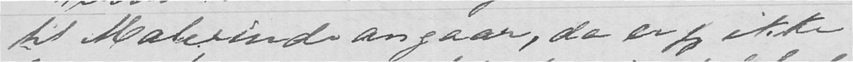til Malerinde angaar, da er jeg ikke
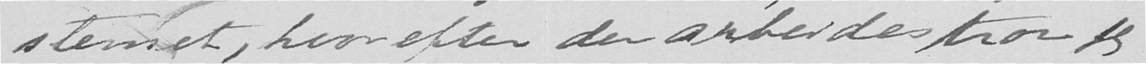stemet, hvor efter der arbeides, tror jeg
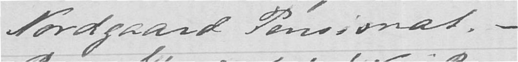Nordgaard Pensionat. -
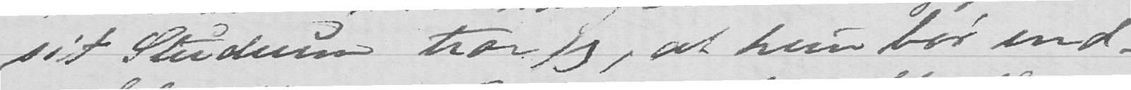sid Studium har jeg, at hun bør ind-
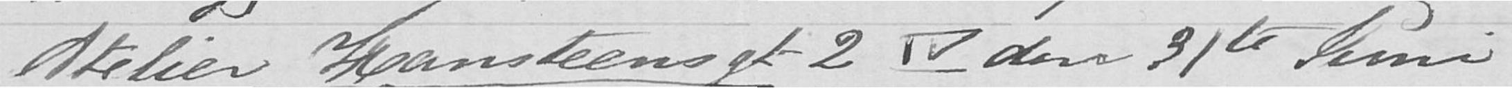Atelier Hansteensgt 2 IV den 31te June
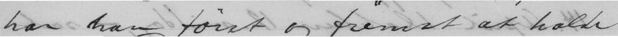har han først og fremst at holde
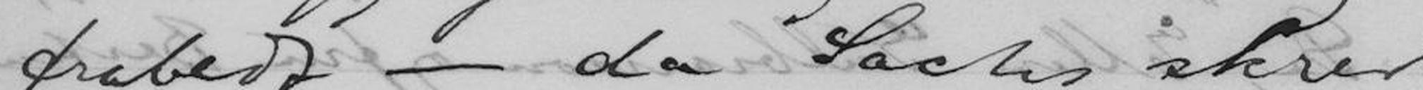frabedt, da Sachs skrev
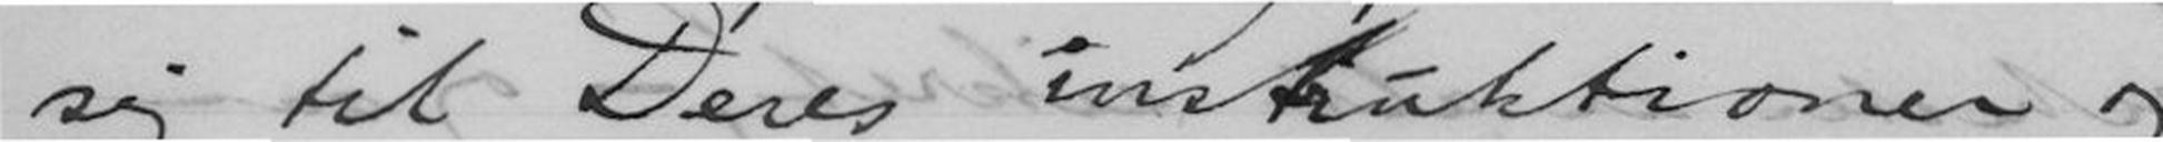sig til Deres instruktioner
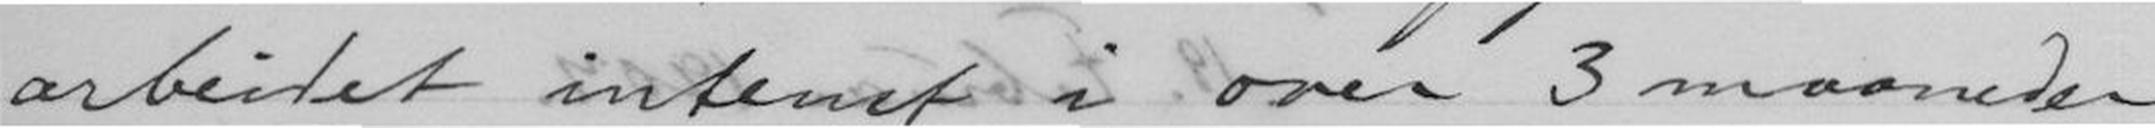arbeidet intenst i over 3 maaneder
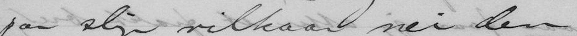paa slige vilkaar, nei den
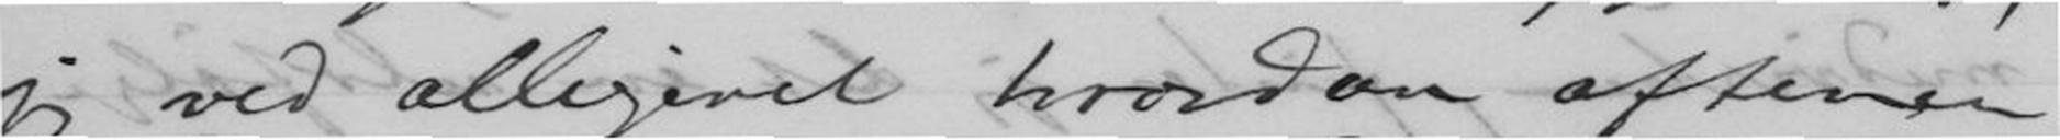jeg ved alligevel hvordan aftenen
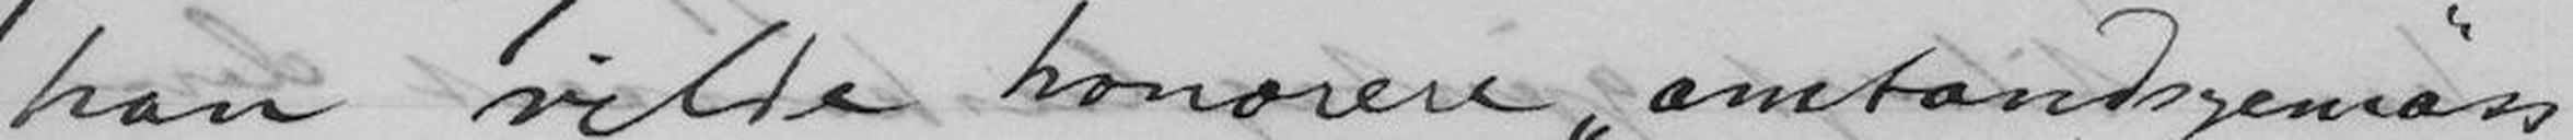han vilde honorere "anstandsgemäss"
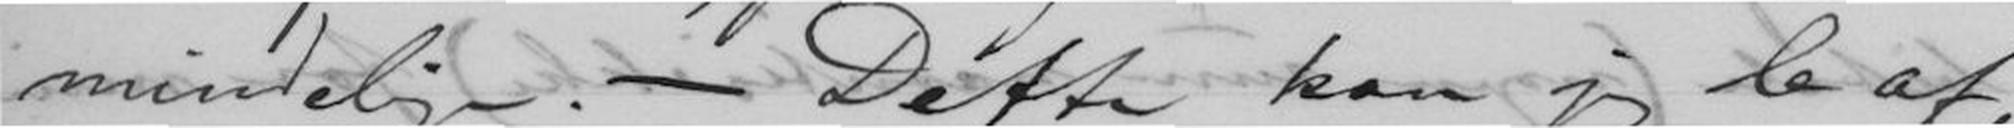mindelige. - Dette kan jeg le af,
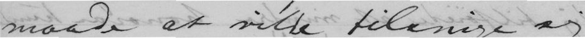maade at vilde tilsnige sig
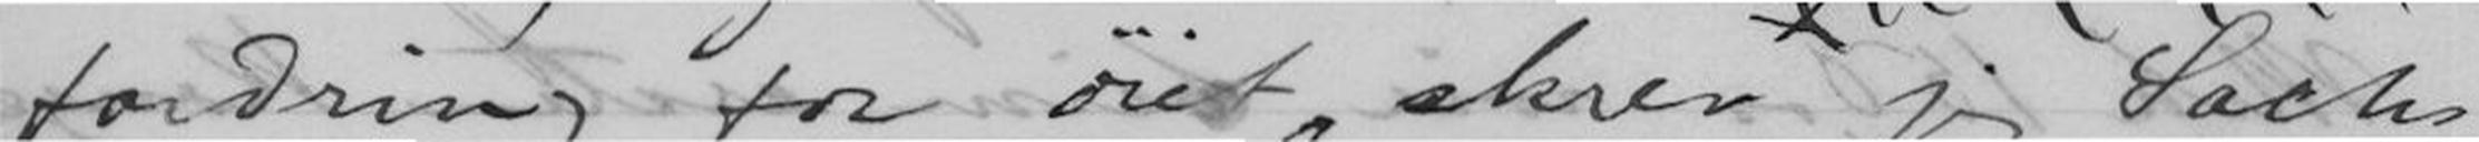fordring for øiet, skrev jeg Sachs
